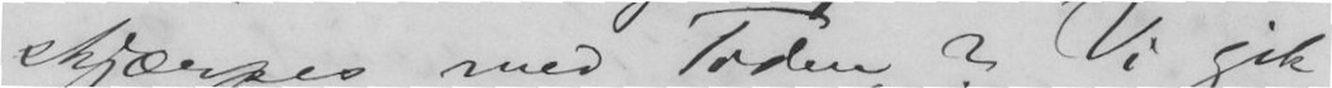skjærpes med Tiden? Vi gik
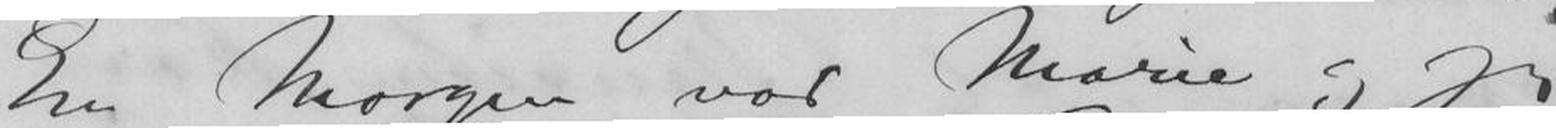En Morgen var Marie og jeg
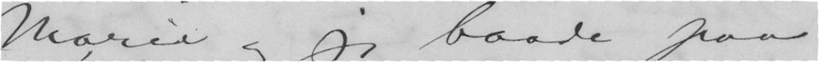Marie og jeg baade paa
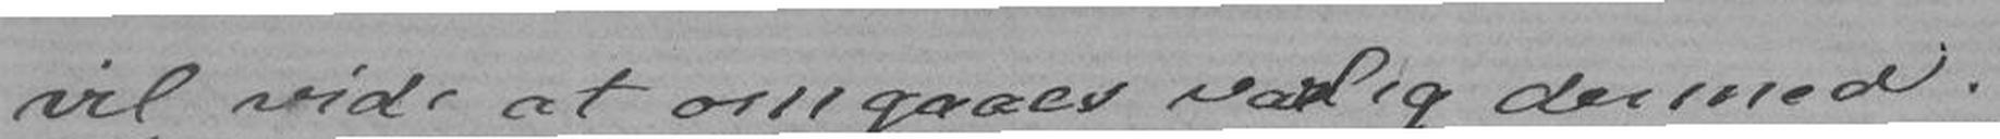vil vide at omgaaes varlig dermed.
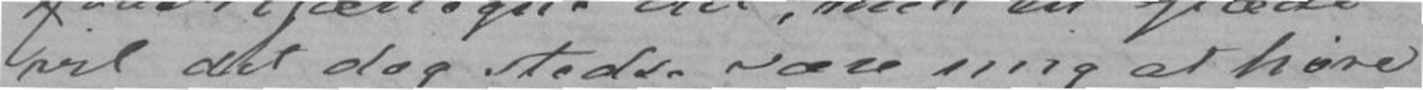vil det dog stedse være mig at høre
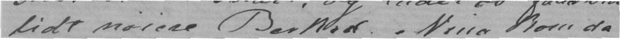lidt nøiere Besked. Nina kom da
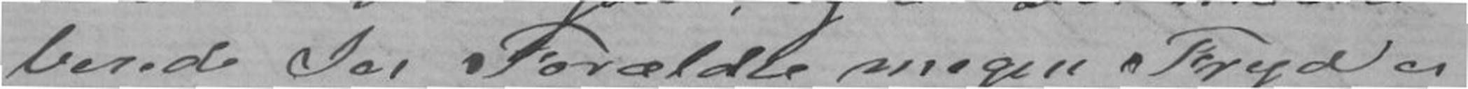berede Jerg Forældre megen Fryd er
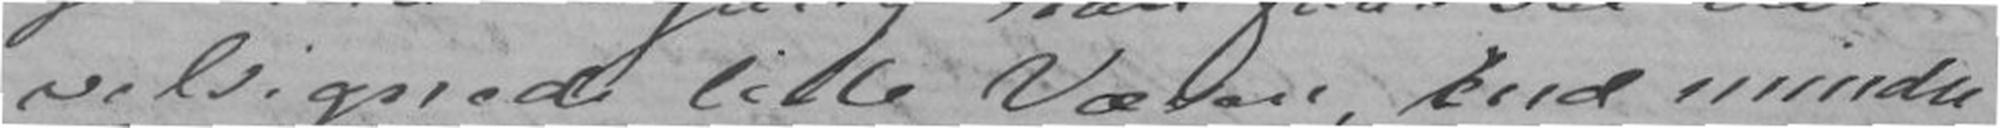velsignede lille Væsen. End mindre
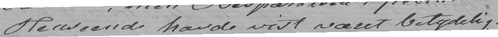Henseende havde vist været betydelig.
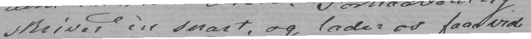skriver Du snart, og lader os faae vide
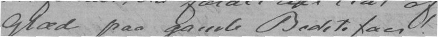glæde paa gamle Bedstefaer!
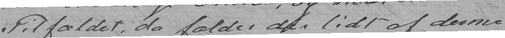Tilfældet da fælder der lidt af denne
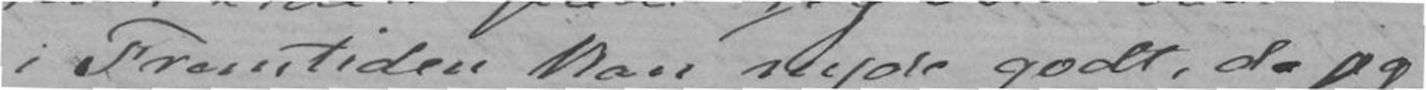i Fremtiden kan nyde godt, da jeg
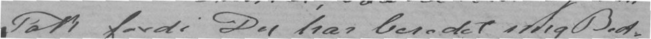Tak fordi Du har beredet mig Bed-
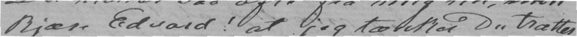kjære Edvard! at jeg tænker Du trættes,
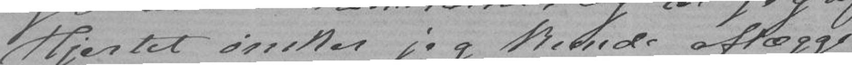Hjertet ønsker jeg kunde aflægge
